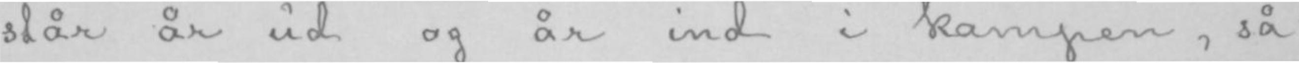står år ud og år ind i kampen, så
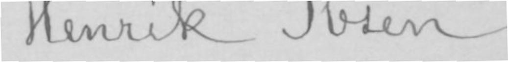Henrik Ibsen.
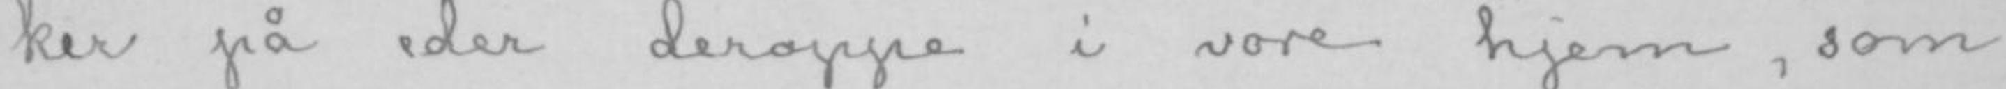ker på eder deroppe i vore hjem, som
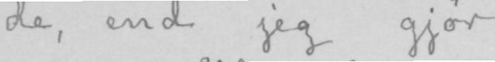de, end jeg gjör.-
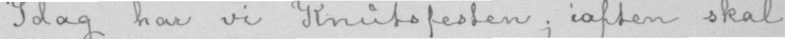Idag har vi Knutsfesten; iaften skal
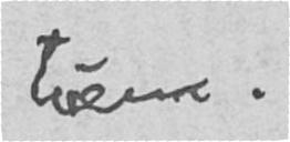tuen.
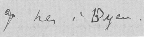op her i Byen.
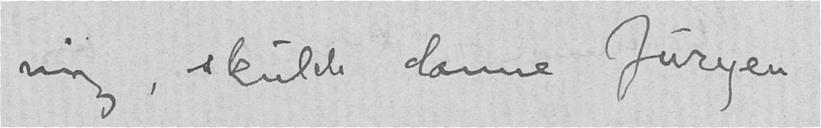ning, skulde danne Juryen.
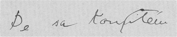De sa komiteen.
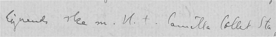lignende ske m.H.t. Camilla Collet Sta-
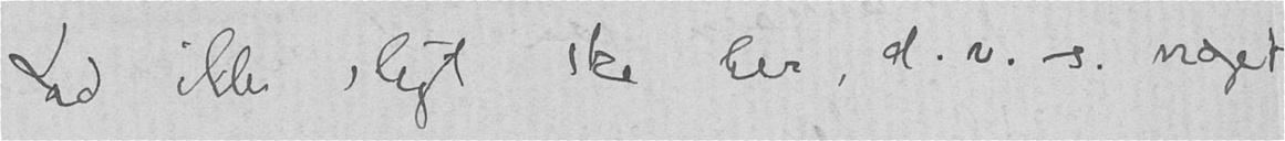Lad ikke sligt ske her, d.v.s. noget
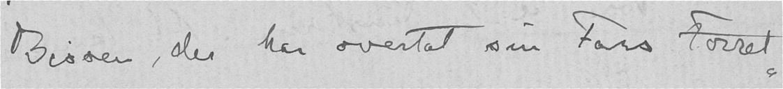Bissen, der har overtat sin Fars Forret-
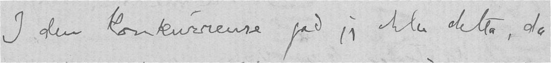I den Konkurrense gad jeg ikke dette, da
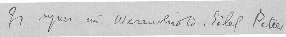Jeg synes nu Werenskiold, Eilef Peter-
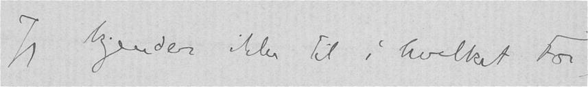Jeg kjender ikke til i hvilket For-
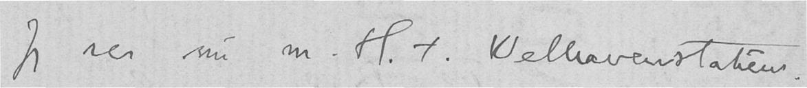Jeg ser nu m.H.t. Welhavenstatuen.
